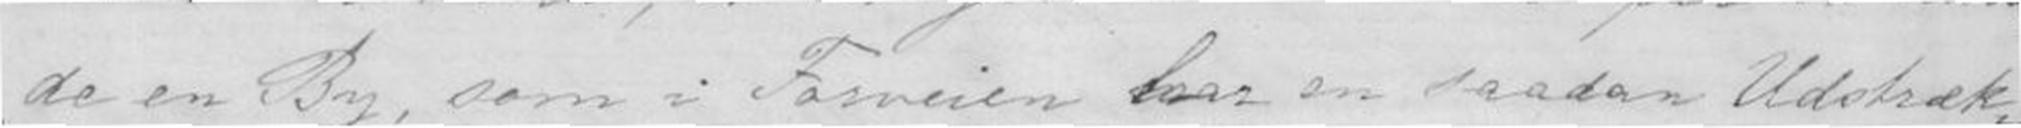de en By, som i Forveien har en saadan Udstræk-
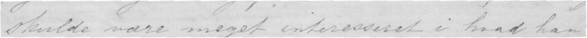skulde være meget interesseret i hvad han
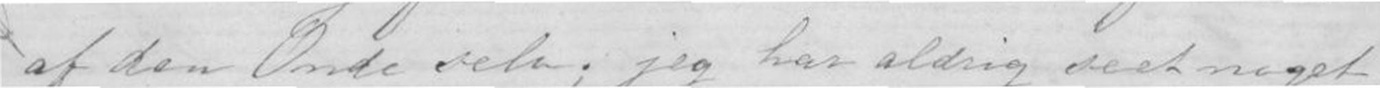af den Onde selv; jeg har aldrig seet noget
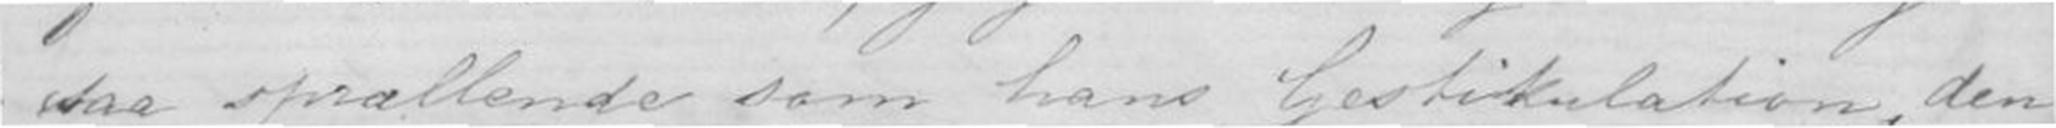saa sprællende som hans Gestikulation, den
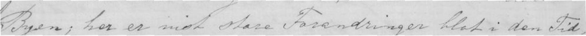Byen; her er vist store Forandringer blot i den Tid,
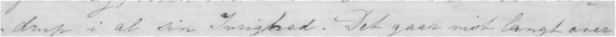drup i al sin Ivrighed. Det gaar vist langt over
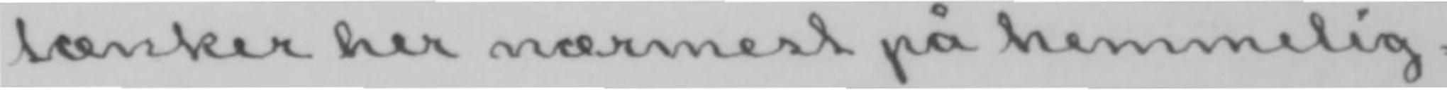tænker her nærmest på hemmelig-
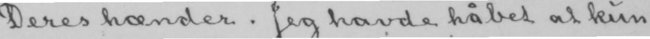Deres hænder. Jeg havde håbet at kun-
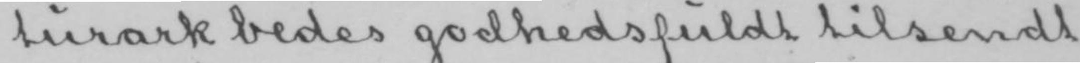turark bedes godhedsfuldt tilsendt
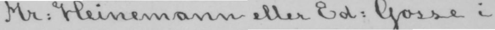Mr: Heinemann eller Ed: Gosse i
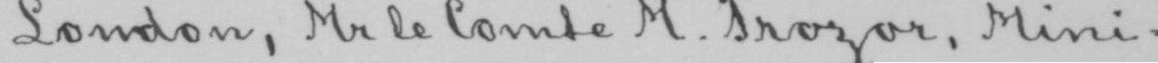London, Mr le Comte M. Prozor, Mini-
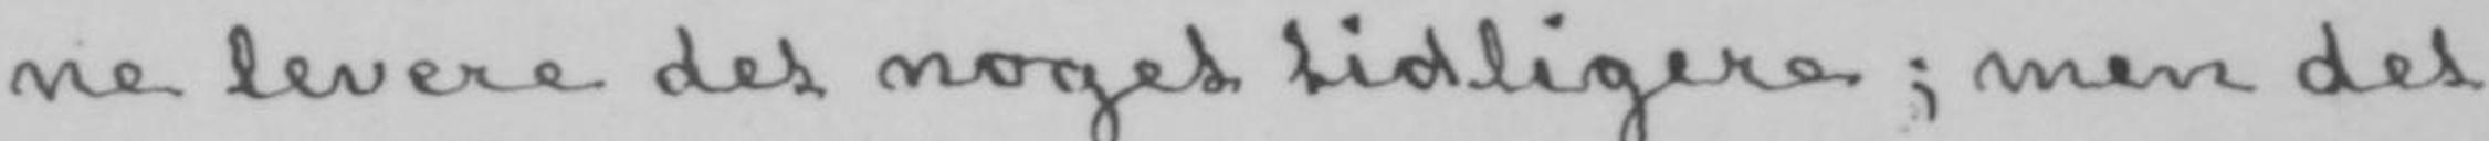ne levere det noget tidligere; men det
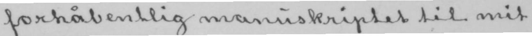forhåbentlig manuskriptet til mit
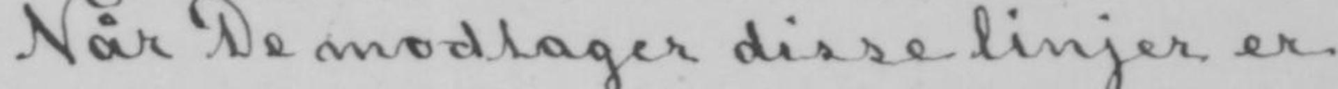Når De modtager disse linjer er
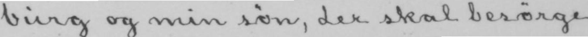burg og min søn, der skal besørge
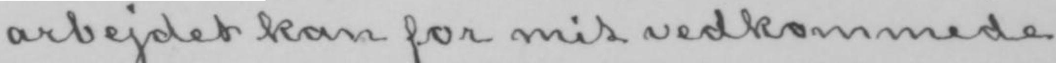arbejdet kan for mit vedkommede
